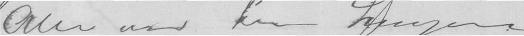Alle ved Din hengivne
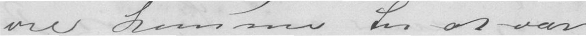vil komme til at vare
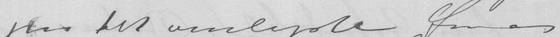paa det venligste fra os
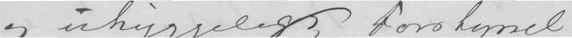og uhyggelig Forstyrrel
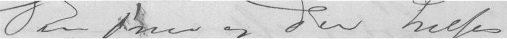Din Frue og Du hilses
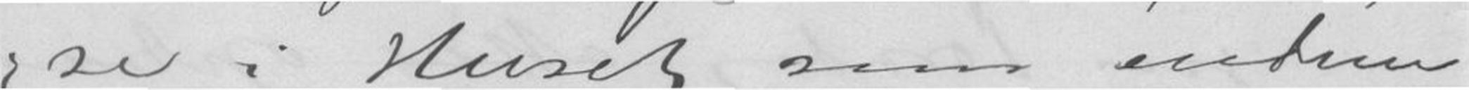se i Huset som endnu
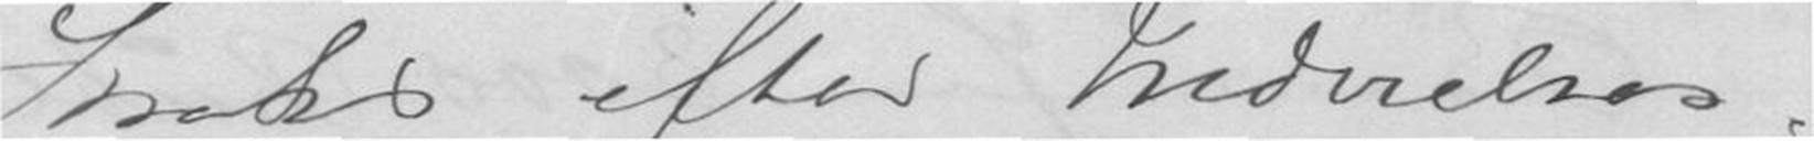Straks efter Indvielses-
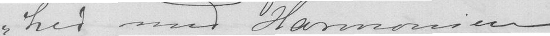hed med Harmonien
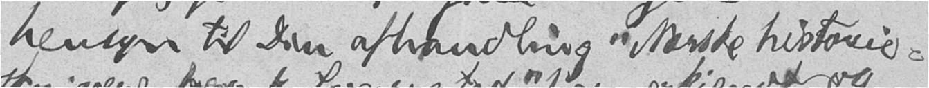hensyn til Din afhandling "Norske historie-
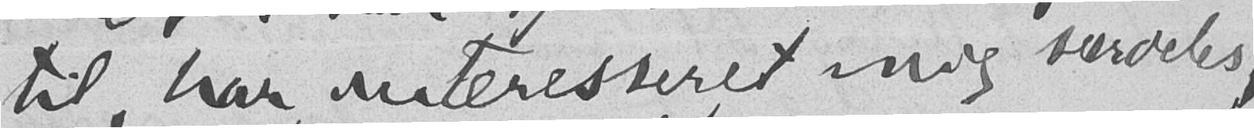til, har interesseret mig særdeles,
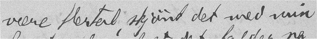være flertal, skjønt det med min
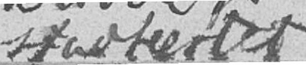stadfæstet.
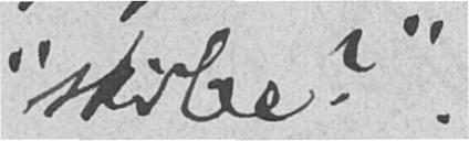"skibe?".
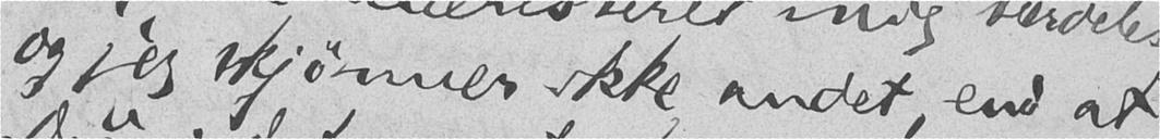og jeg skjønner ikke andet, end at
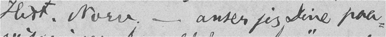Hist. Norv. - anser jeg Dine paa-
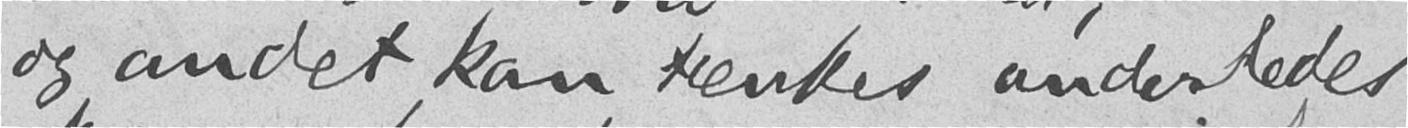og andet kan tænkes anderledes.
